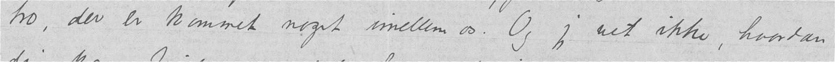tro, der er kommet noget imellem os. Og jeg vet ikke, hvordan
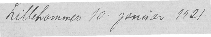Lillehammer 10. januar 1921.
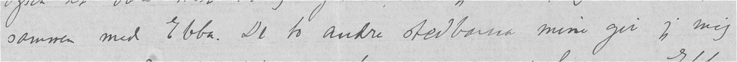sammen med Ebba. De to andre stedbarna mine gir jeg mig
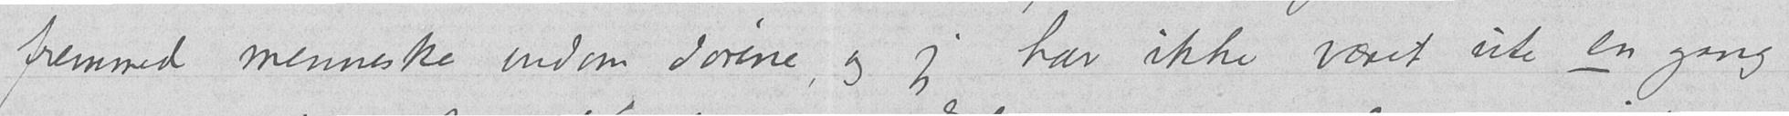fremmed menneske indom dørene, og jeg har ikke været ute en gang
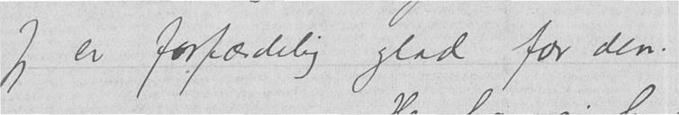Jeg er forfærdelig glad for den.
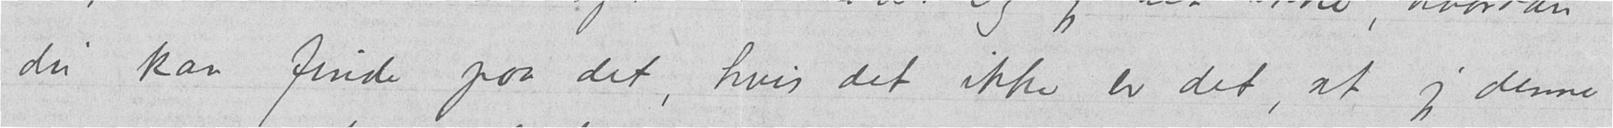du kan finde paa det, hvis det ikke er det, at jeg denne
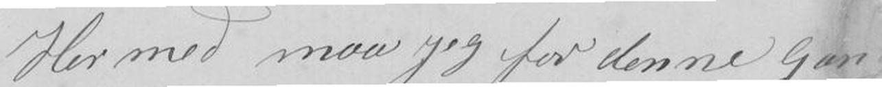Hermed maa jeg for denne Gang
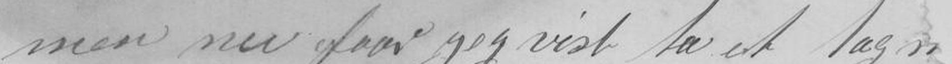men nu faar jeg vist ta et tag
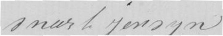snart gjensyn
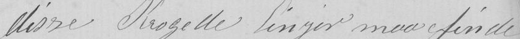disse Krogede Linjer maa finde
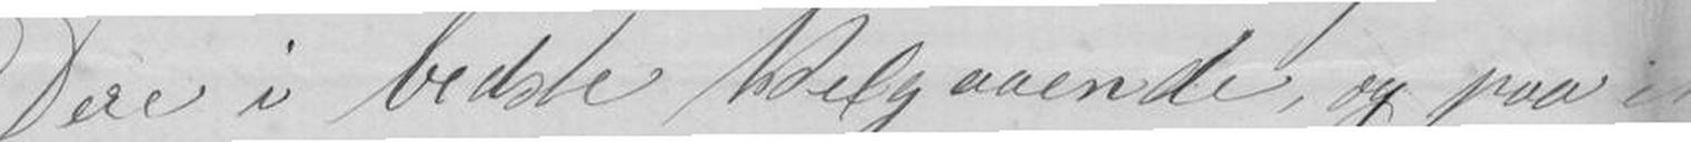Dere i bedste Velgaaende, og paa et
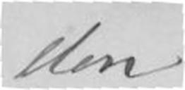den -
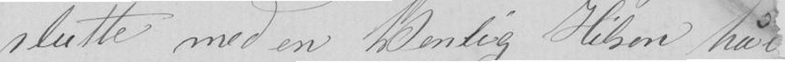slutte med en Venlig Hilsen har
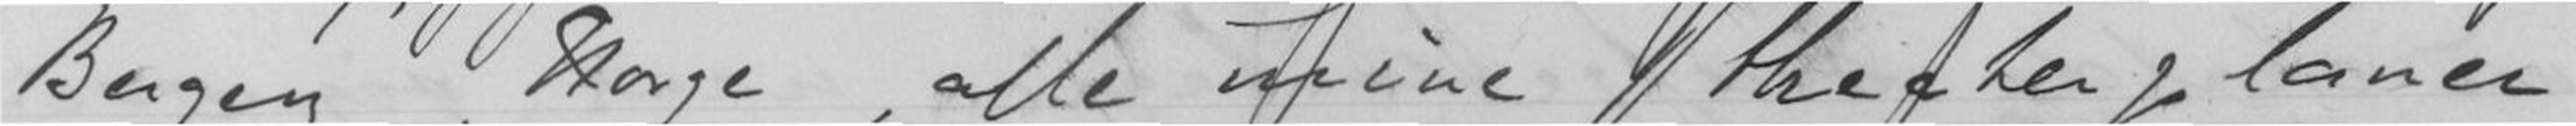Bergen, Norge, alle mine theaterplaner
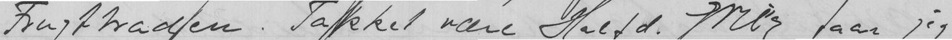Fragttraden. Takket være Halfd. Grieg faar jeg
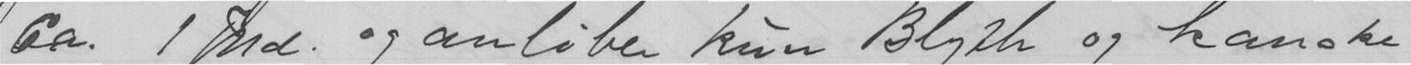Ca. 1 Md. og anløber kun Blyth og kanske
